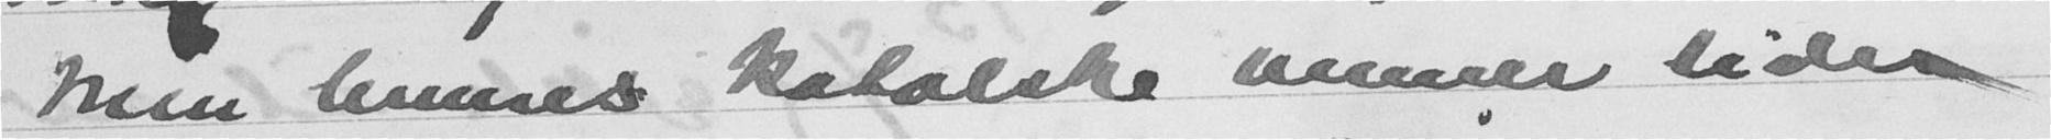Men hennes katolske venner lider
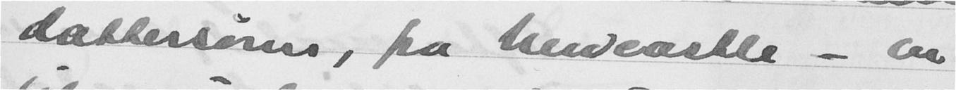dattersønn, fra Marseaelle - en
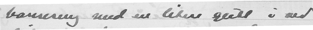barneseng med en liten gutt i ved
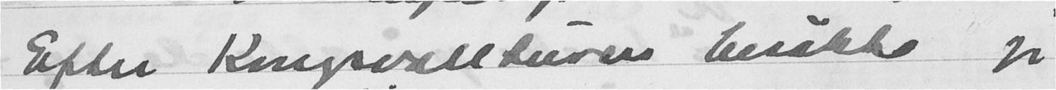Efter Kongsvollturen besøkte jeg
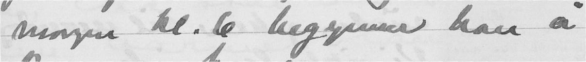morgen kl. 6 begynner han å
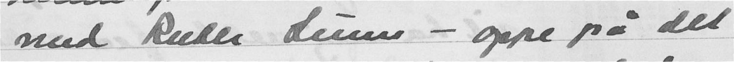med Ruth Leum - oppe på det
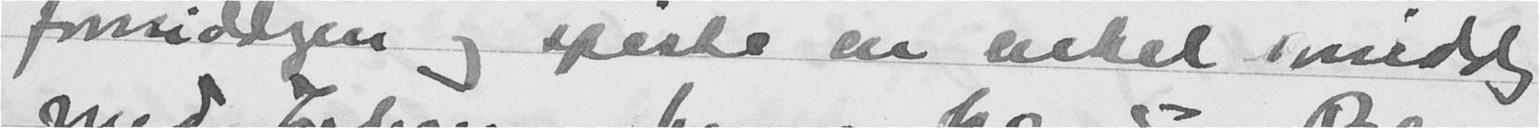formiddagen og spiste en enkel middag
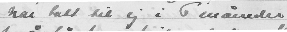har tatt til sig i 5 måneder
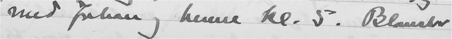med Johan og henne kl. 5. Blansbor
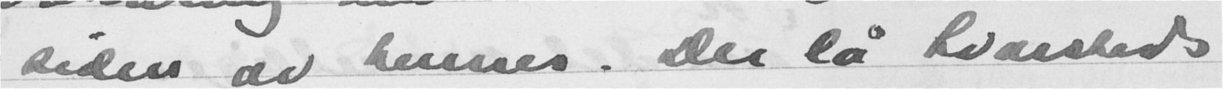siden av hennes. Der la Svarstads
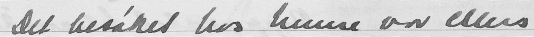Det besøket hos henne var ellers
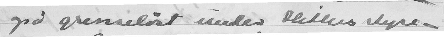også grenseløst under Hitlers styre.
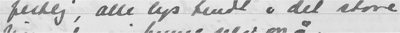festlig, alle lys tendt i det store
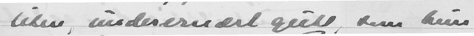liten underernært gutt, som hun
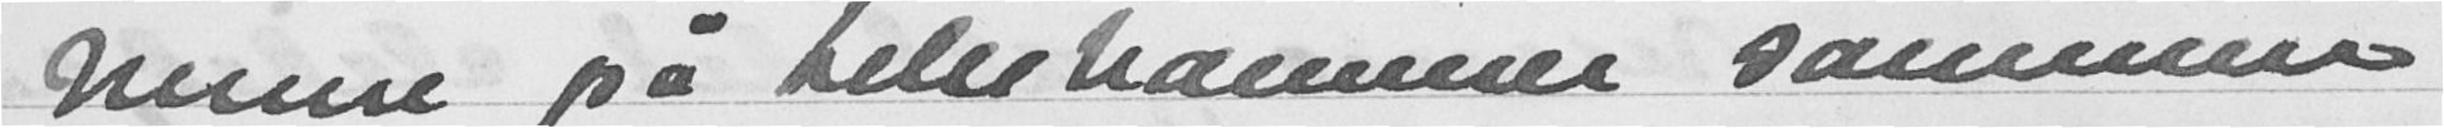henne på Lillehammer sammen
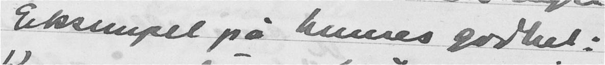Eksempel på hennes godhet:
